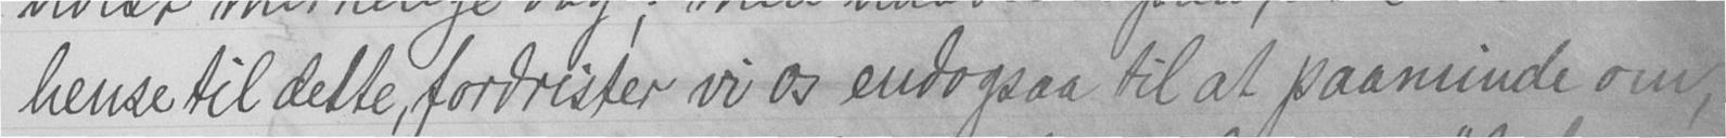hense til dette, fordrister vi os endogsaa til at paaminde om,
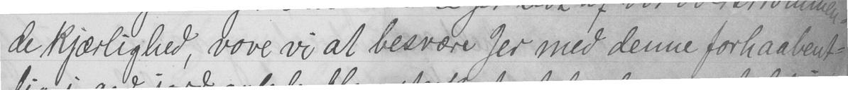de kjærlighed, vove vi at besvære Jer med denne forhaabent-
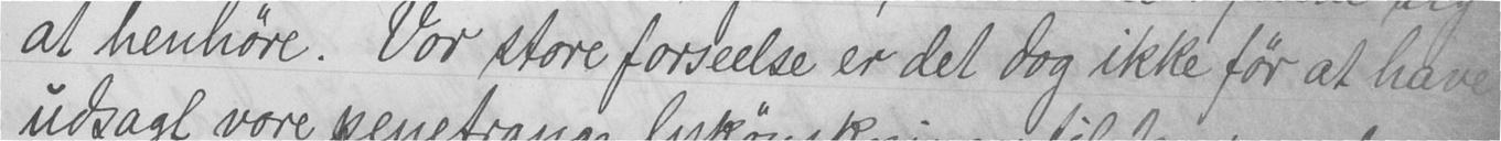at henhøre. Vor store forseelse er det dog ikke før at have
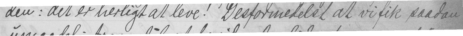den: det er herligt at leve! Desformedelst at vi fik saadan
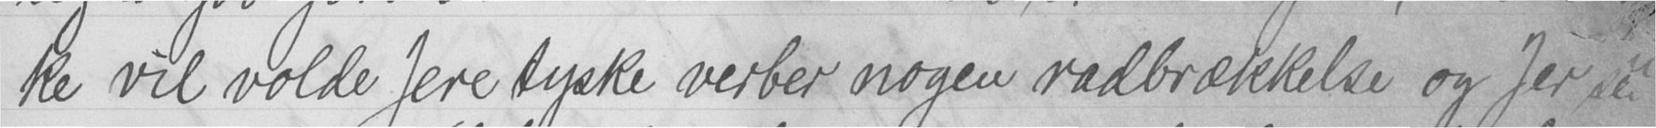ke vil volde Jere tyske verber nogen radbrækkelse og Jer se
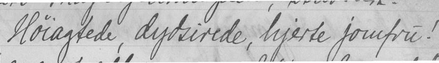Høiagtede, dydsirede, hjerte jomfru!
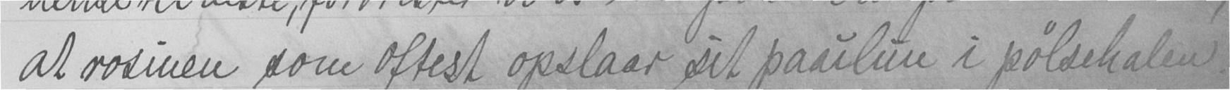at rosinen som oftest opslaar sit paulun i pølsehalen.
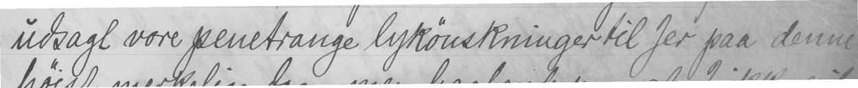udsagt vore penetrange lykønskninger til Jer paa denne
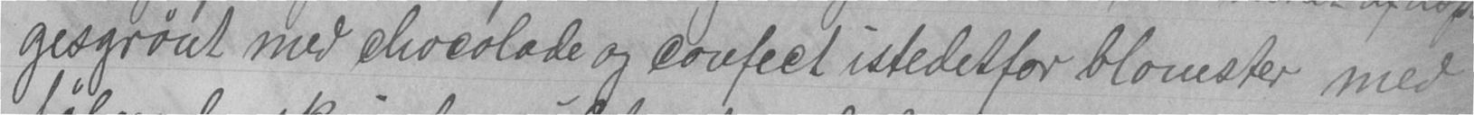gesgrønt med chocolade og confect istedetfor blomster med
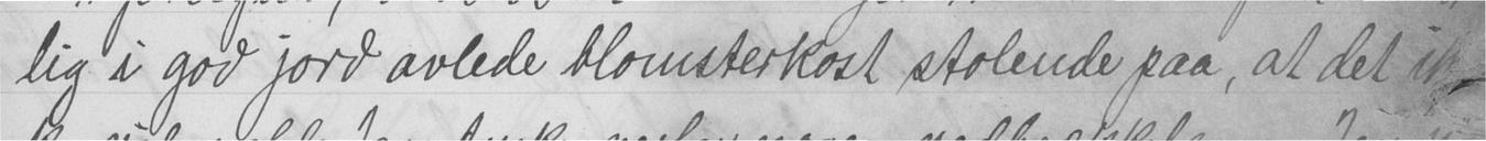lig i god jord avlede blomsterkost stolende paa, at det ik-
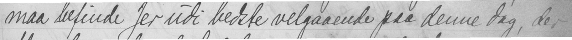maa befinde Jer udi bedste velgaaende paa denne dag, der
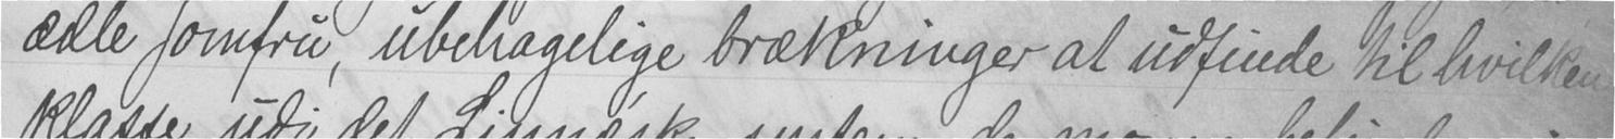ædle jomfru, ubehagelige brækninger at udfinde til hvilken
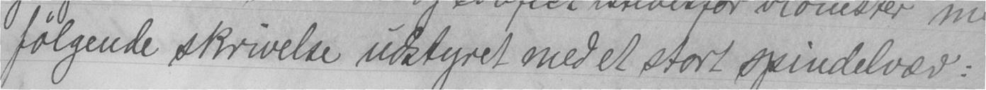følgende skrivelse udstyret med et stort spindelvæd:
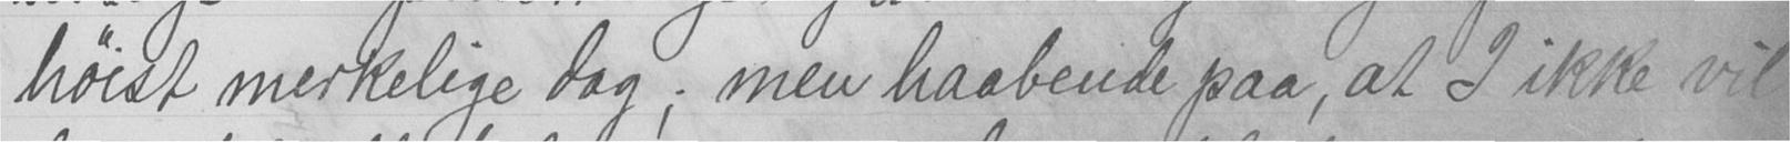høist merkelige dag; men haabende paa, at I ikke vil
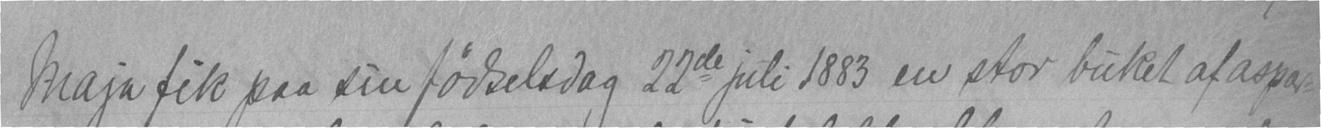Maja fik paa sin fødselsdag 22de juli 1883 en stor buket af aspar-
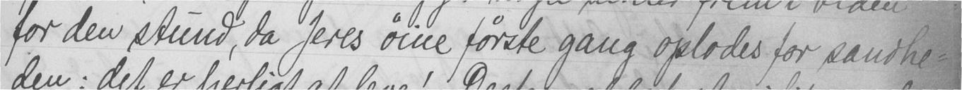for den stund, da Jeres øine første gang oplodes for sandhe-
